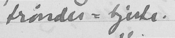trønder-hjerte.
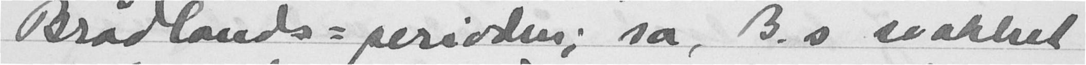Brådlands-perioden; sa, B.s svakhet
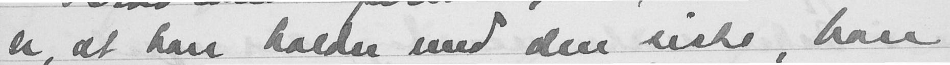er, at han holder med den siste, han
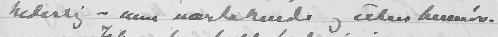hederlig - men nærtakende og uten honnør.
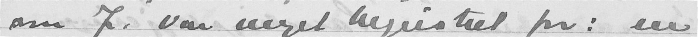som J. var meget begeistret for: en
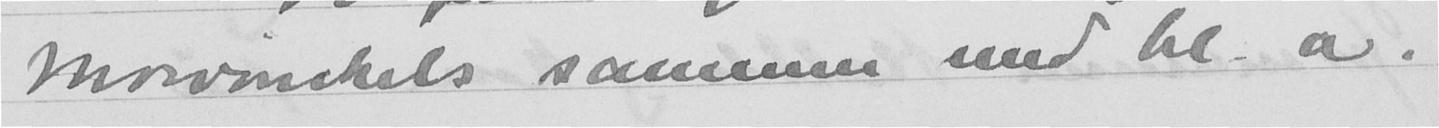Mowinckels sammen med bl. a.
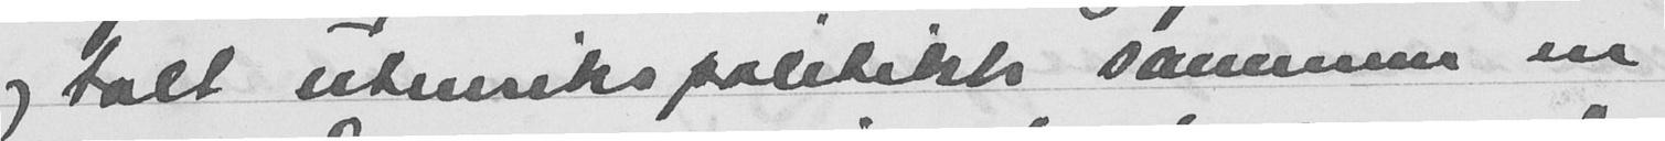og talt utenrikspolitikk sammen en
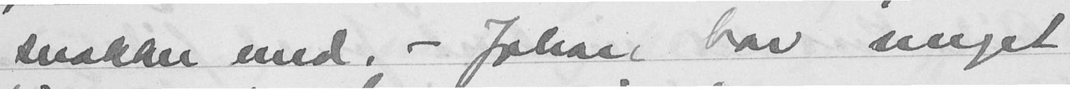snakker med. - Johan har meget
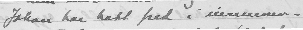Johan har hatt fred i vinmono-
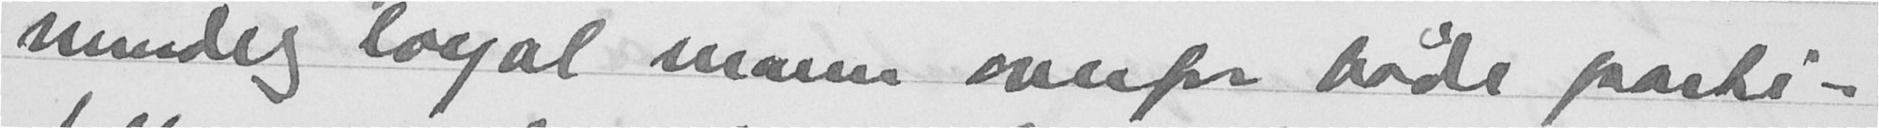mindelig lojal mann overfor bade parti-
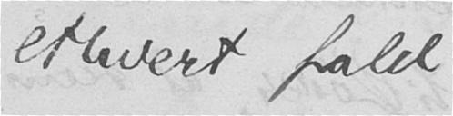ethvert fald
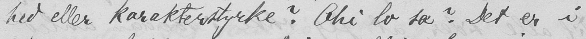hed eller karakterstyrke? Chi lo sa? Det er i
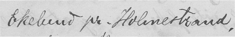Ekelund pr. Holmestrand,
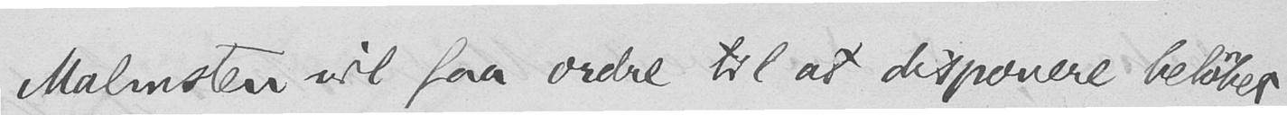Malmsten vil faa ordre til at disponere beløbet,
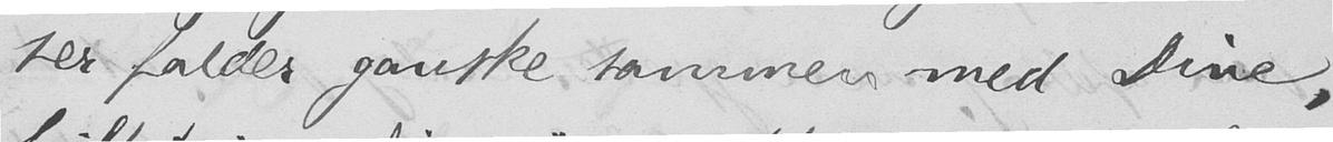ser falder ganske sammen med Dine,
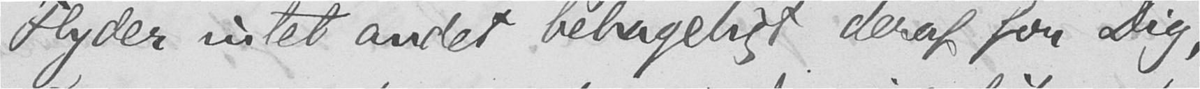Flyder intet andet behageligt deraf for Dig,
